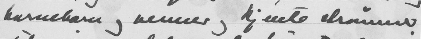barnebarn og venner og kjente strømmet
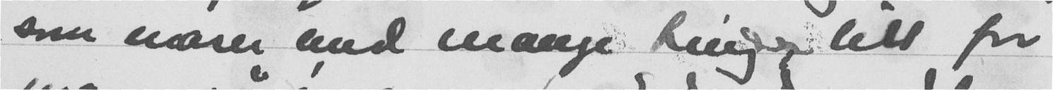som nærer med mange ting  litt for
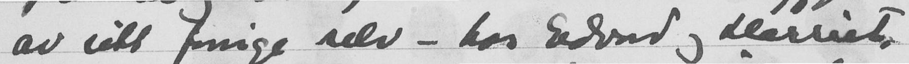av sitt forrige selv - hos Edvard og Harriet,
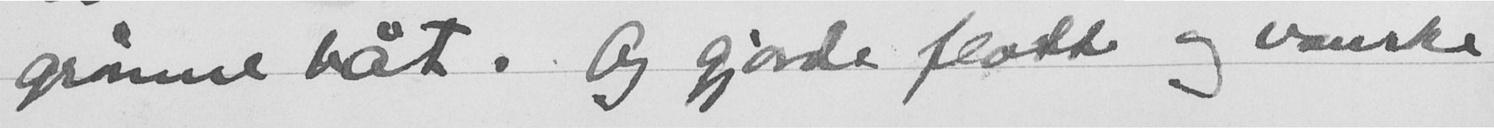grønne båt. Og gjorde flotte og vanske
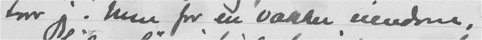tror jeg. Men for en vakker eiendom!
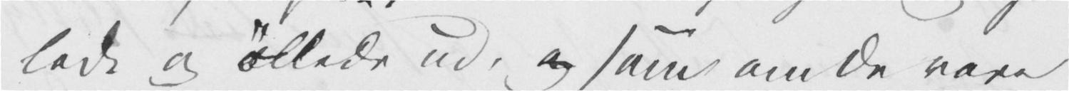lede og øllede ud, og som om de vare
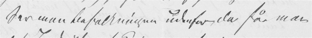Ser man Befolkningen udenfor, da får man
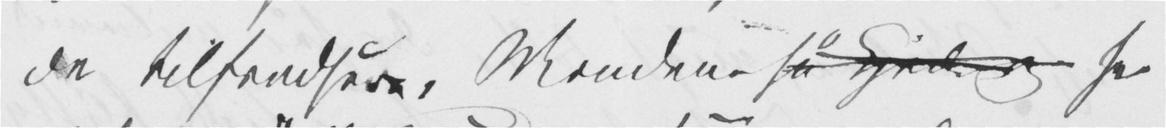de tilfredsere, Mændene se kjede sig ser
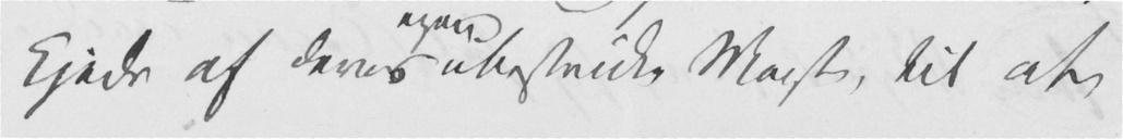kjede af deres ubestridte Magt, til at
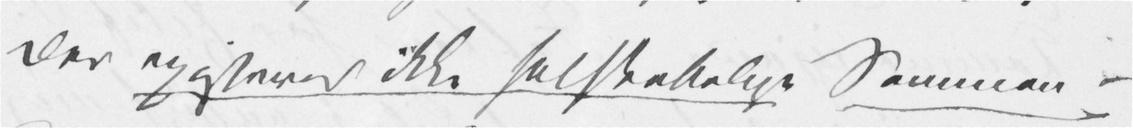Der existerer ikke selskabelige Sammen-
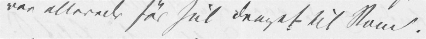var allerede før Jul draget til Rom.
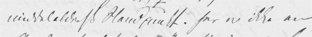middelaldersk Standpunkt. her er ikke en
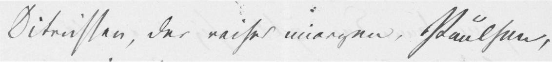Ditrichsen, der reiser imorgen, Paulsen,
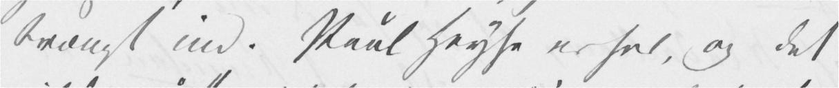trængt ind. Paul Heyse er her, og det
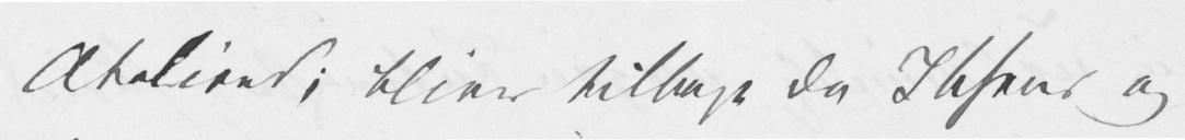Atelier; bliver tilbage da Ibsens og
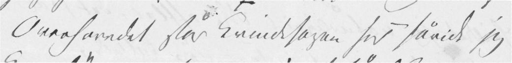Overhovedet står Kvindesagen her såvidt jeg
